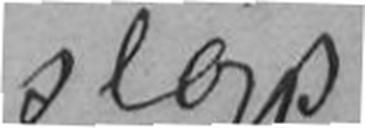slags
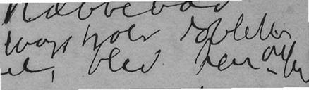tvagstrolr doblellr alber
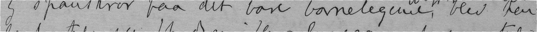og spanskrør paa det bare barnelegene, blev der
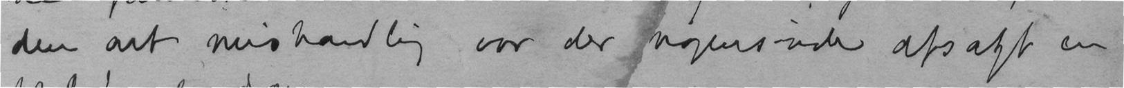den art mishandling var der nogensinde afsagt en
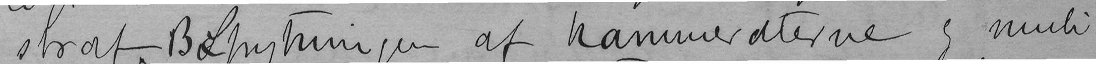straf. Bælpytningen af kammeraterne og muli-
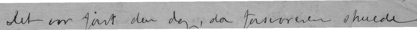Det var først den dag, da forsvareren skulde
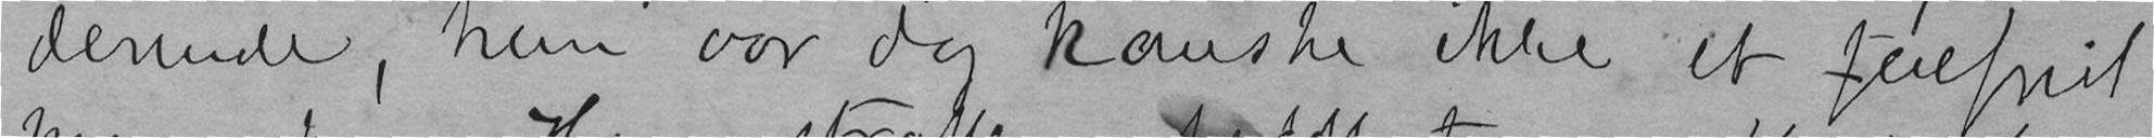derinde, hun var dog kanske ikke et feilfrit
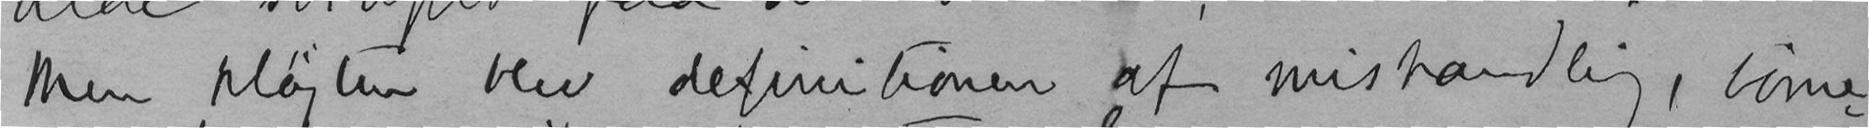Men pligten blev definitionen af mishandling, børne-
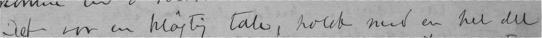Det var en kløgtig tale, holdt med en hel del
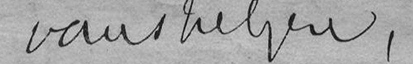vanskeligere,
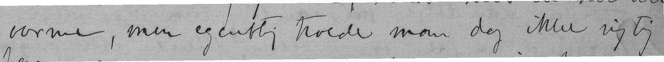varme, men egentlig troede man dog ikke rigtig
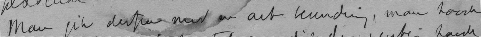Man gik derfra med en art undring, man havde
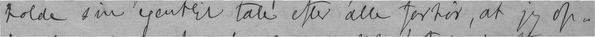holde sin egentlige tale efter alle forhør, at jeg op-
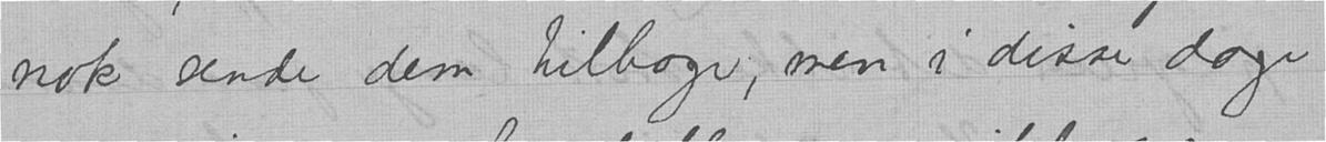nok sende den tilbage, men i disse dage
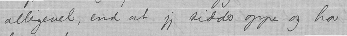alligevel, end at jeg sidder oppe og har
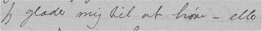Jeg gleder meg til at høre - eller
